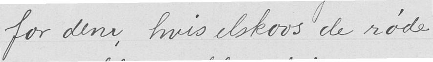for dem, hvis elskovs de røde
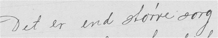Det er end større sorg
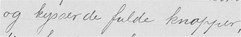og kysser de fulde knopper,
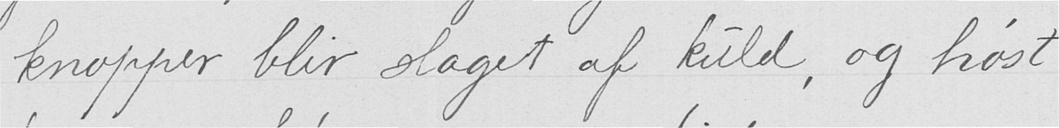knopper blir slaget af kuld, og høst
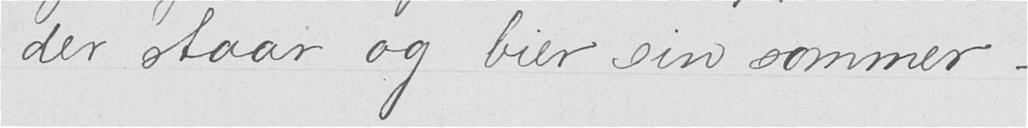der staar og bier sin sommer - -.
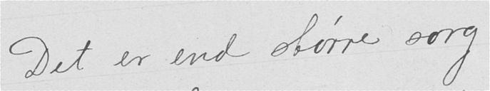Det er end større sorg,
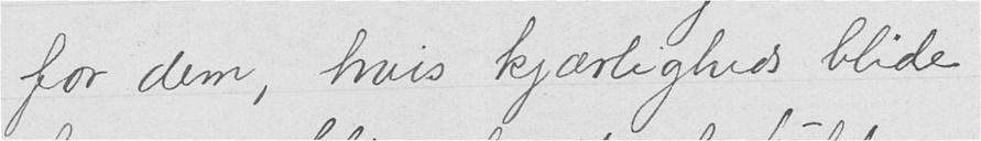for dem, hvis kjærligheds blide
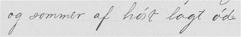og sommer af høst lagt øde.
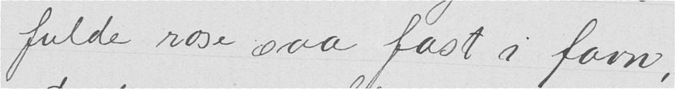fulde rose saa fast i favn,
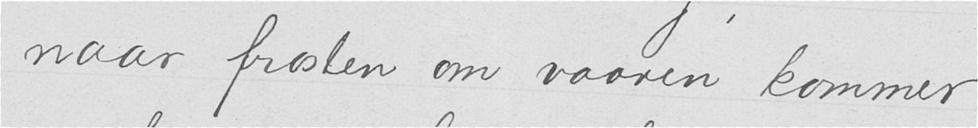naar frosten om vaaren kommer
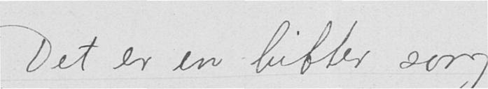Det er en bitter sorg -
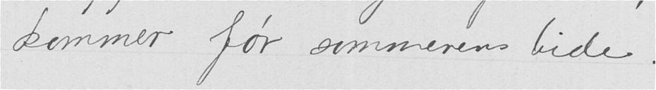kommer før sommerens tide.
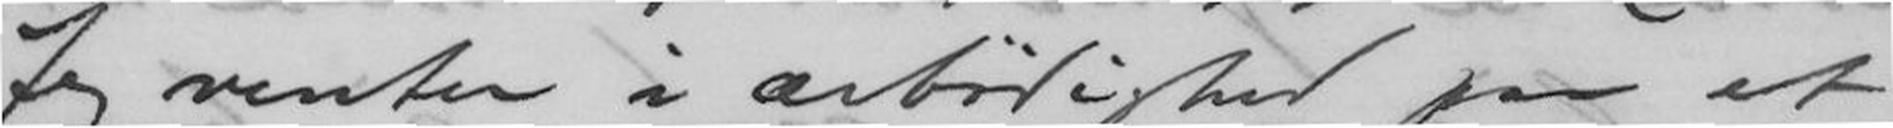Jeg venter i ærbødighed paa et
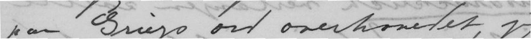paa Griegs ord overhovedet, jeg
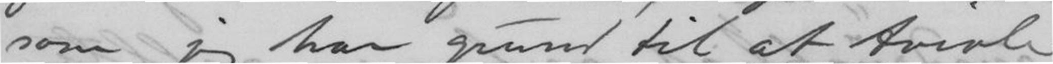som jeg har grund til at tvivle
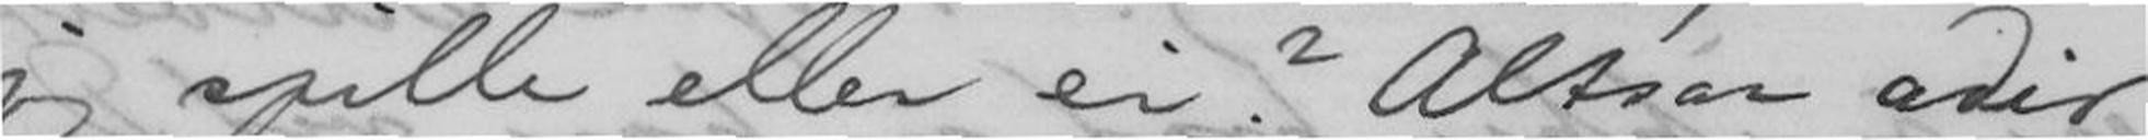jeg spille eller ei? Altsaa adir
